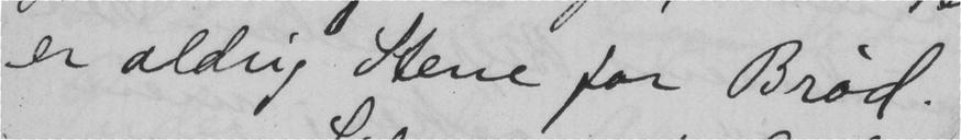er aldrig Stene for Brød.
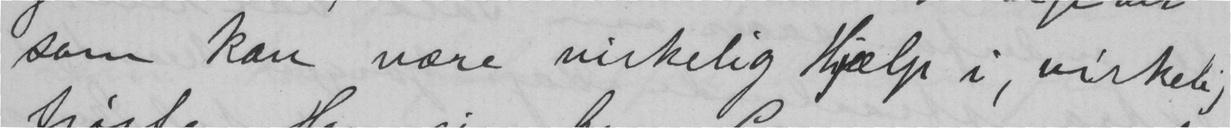som kan være virkelig Hjælp i, virkelig
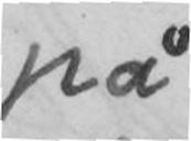på
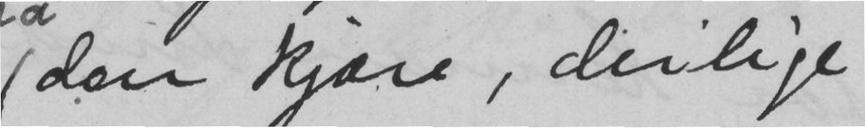den kjære, deilige
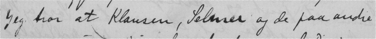Jeg tror at Klausen, Selmer og de faa andre
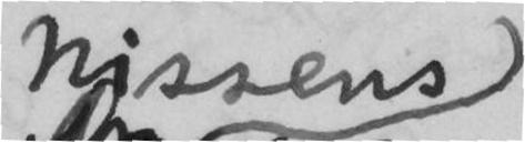Nissens
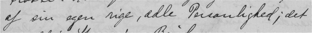af sin egen rige, ædle Personlighed; det
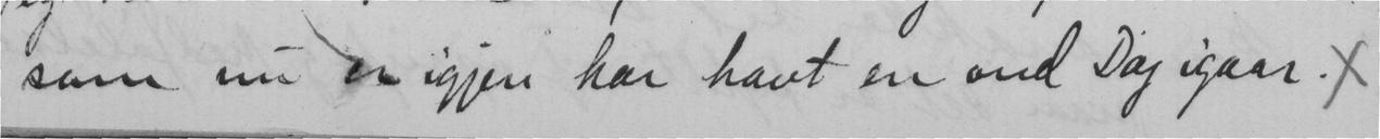som nu er igjen har havt en ond Dag igaar.x
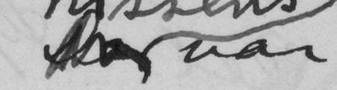var
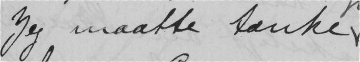Jeg maatte tænke
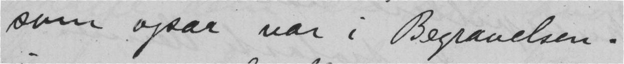som ogsaa var i Begravelsen. Dog var
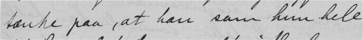tænke paa, at han som hun hele
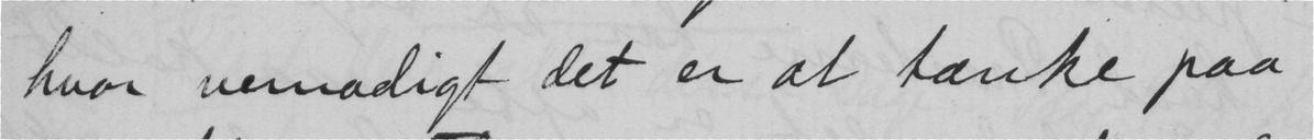hvor vemodigt det er at tænke paa
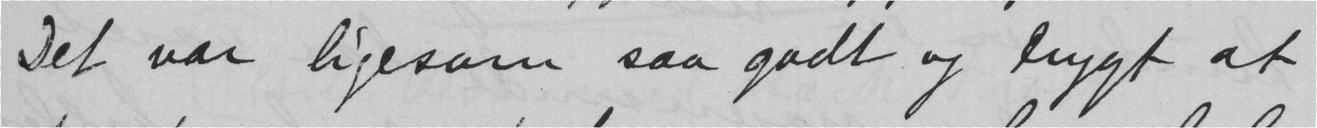Det var ligesom saa godt og trygt at
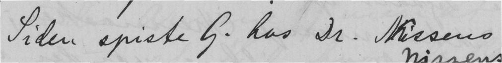Siden spiste G. hos Dr. Nissens
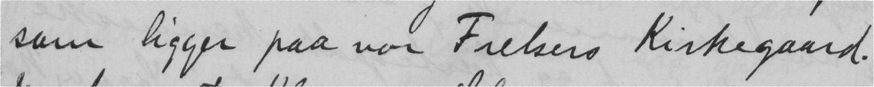som ligger paa vor Frelsers Kirkegaard.
